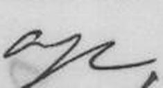op,
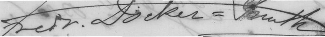Fredr. Döcker=Smith.
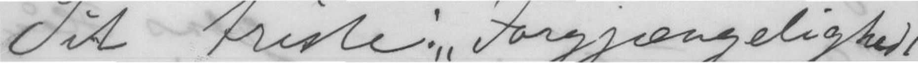Sit triste: "Forgjængelighed!"
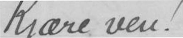Kjære ven!
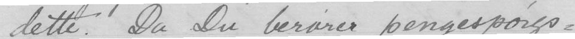dette. Da Du berører pengespørgs-
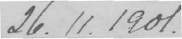26.11.1901.
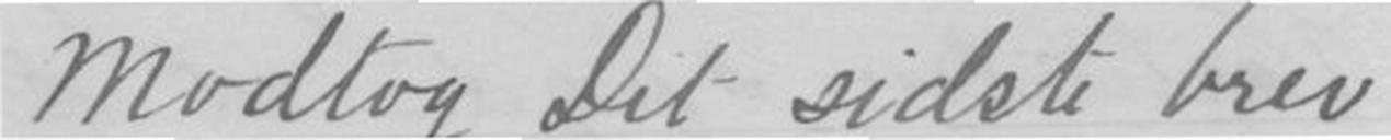Modtog Dit sidste brev
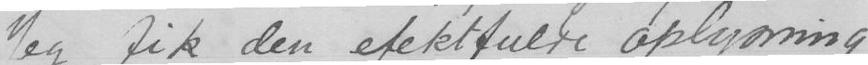Jeg fik den efektfulde oplysning
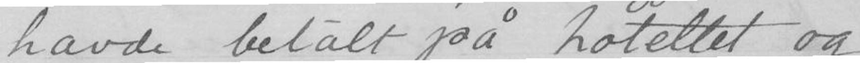havde betalt på hotellet og
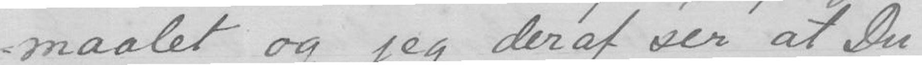maalet og jeg deraf ser at Du
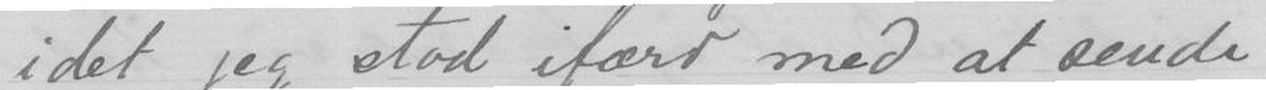idet jeg stod ifærd med at sende
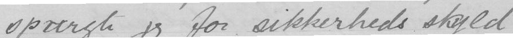spurgte jeg for sikkerheds skyld
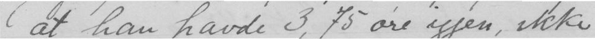at han havde 3,75 øre igjen, ikke
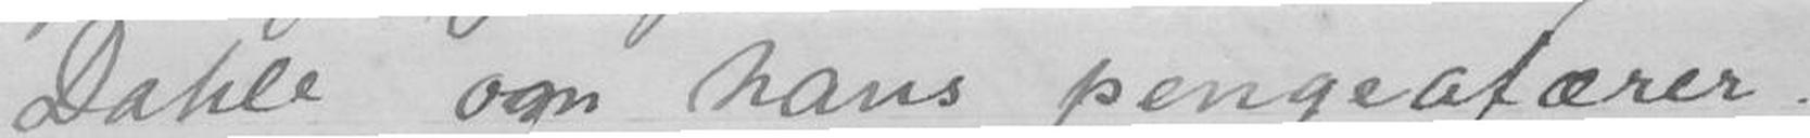Dahle om hans pengeafærer.
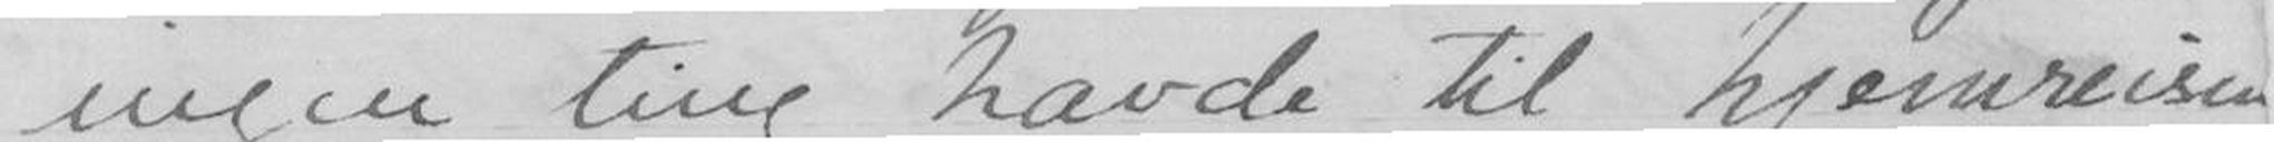ingen ting havde til hjemreisen.
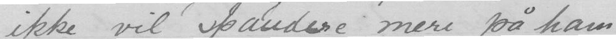ikke vil spandere mere på ham
